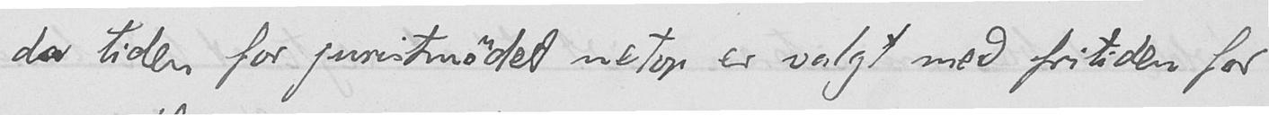da tiden for juristmødet netop er valgt med fritiden for
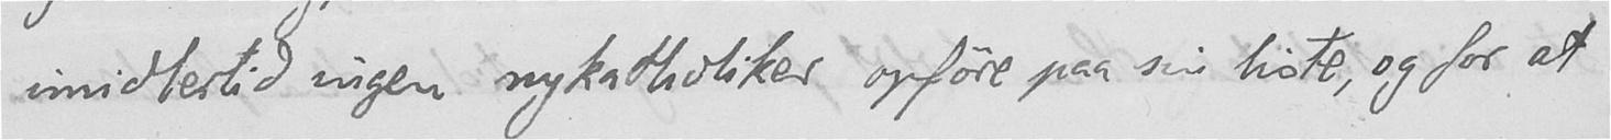imidlertid ingen nykatholiker opføre paa sin liste, og for at
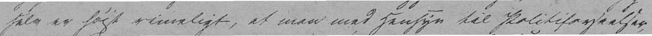selv er høist rimeligt, at man med Hensyn til Politiforseelser,
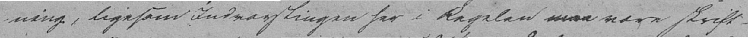ning, ligesom Indvarslingen her i Regelen maa være skrift-
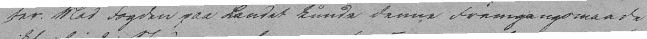ter. Mod Fogden paa Landet kunde denne Fremgangsmaade
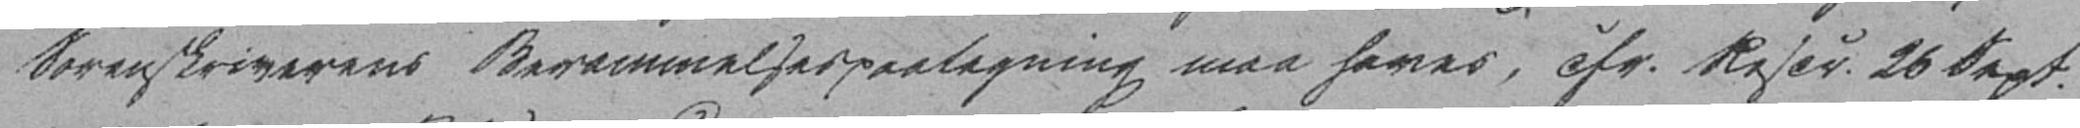Sorenskriverens Berammelsespaategning maa haves, cfr. Rescr. 26 Sept.
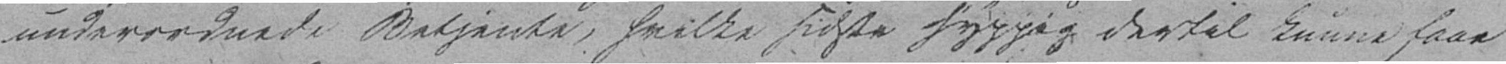underordnede Betjente, hvilke sidste hyppig dertil kunne faae
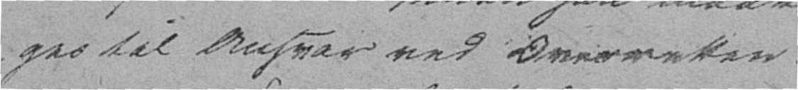ges til Ansvar ved Overretten.
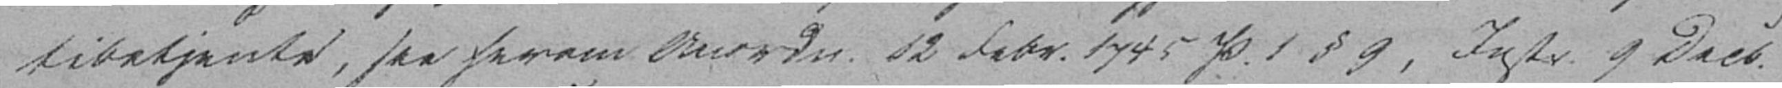tibetjente, see herom Anordn. 12 Febr. 1745 P. 1 § 9, Instr. 9 Decb.
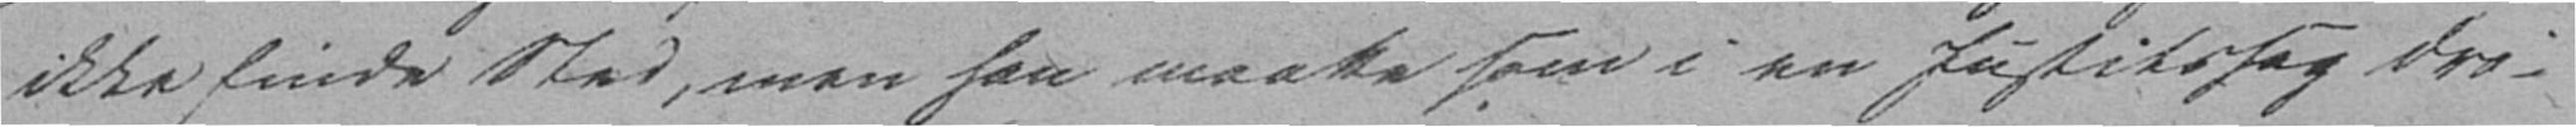ikke finde Sted, men han maatte som i en Justitssag dra-
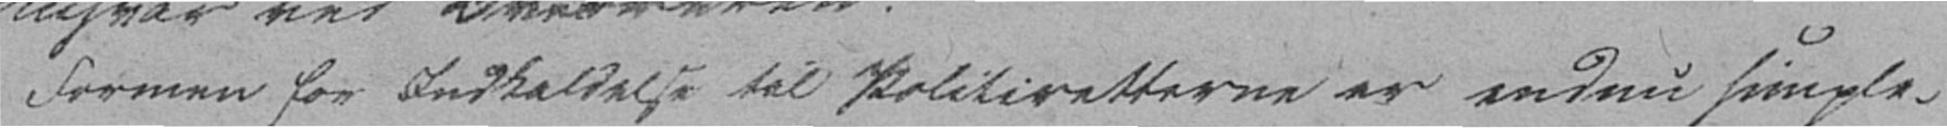Formen for Indkaldelse til Politiretterne er endnu simple-
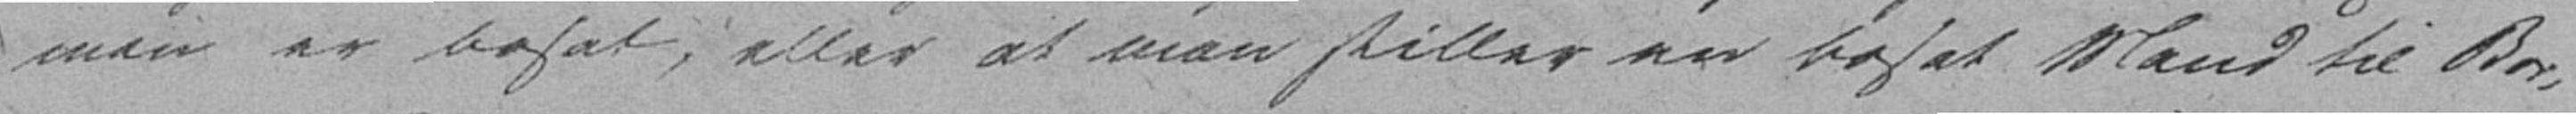man er bosat, eller at man stiller en bosat Mand til Bor-
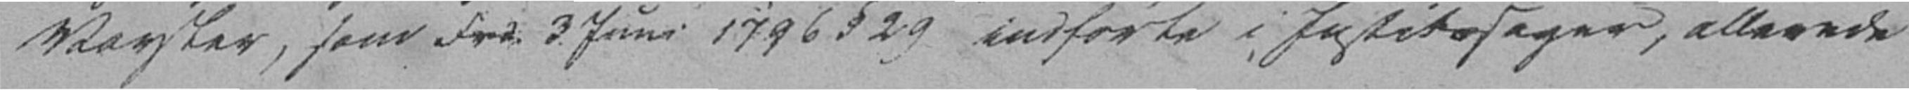Varsler, som Frd. 3. Juni 1796 § 29 indførte i Justitssager, allerede
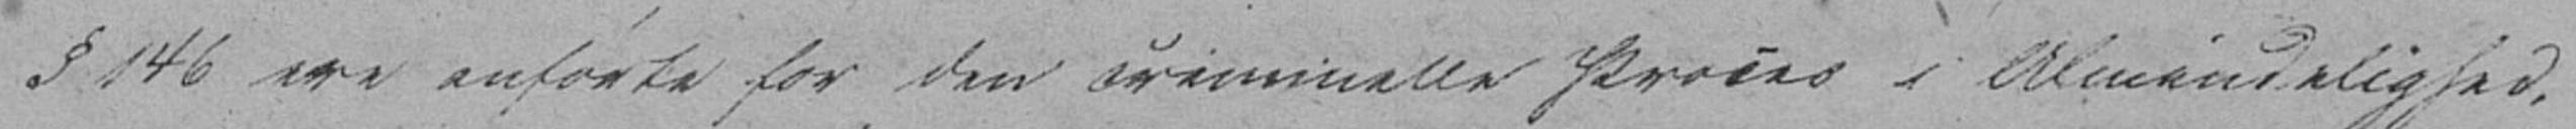§ 146 ere anførte for den criminelle Proces i Almindelighed.
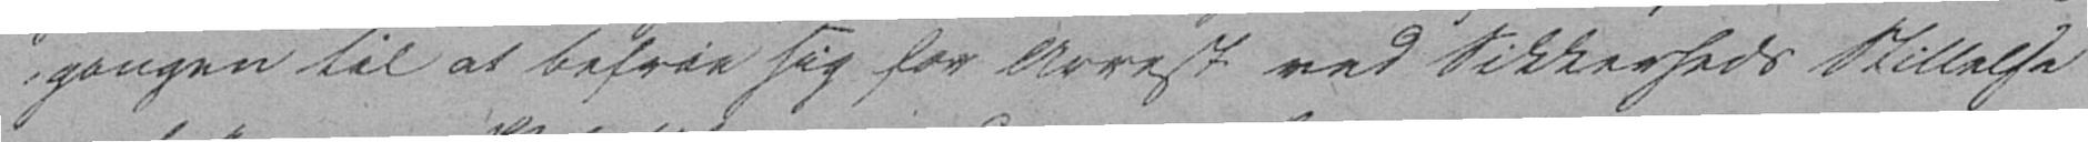gangen til at befrie sig for Arrest ved Sikkerheds Skillelse
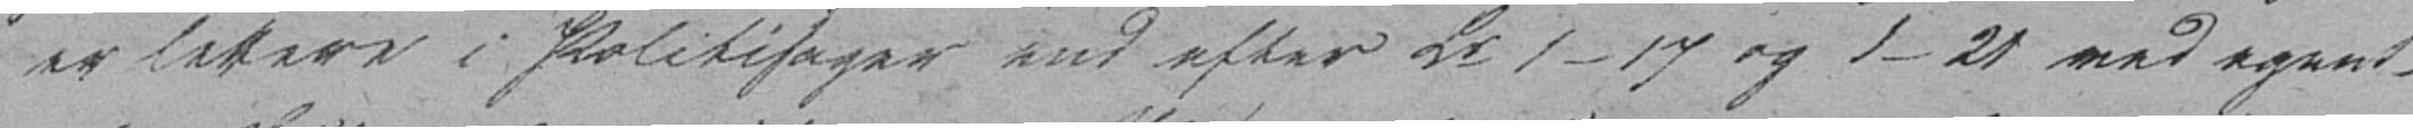er lettere i Politisager end efter L. 1-17 og 1-21 ved egen-
